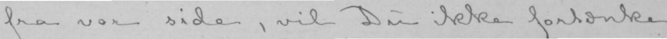fra vor side, vil Du ikke fortænke
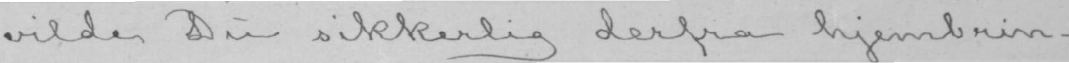vilde Du sikkerlig derfra hjembrin-
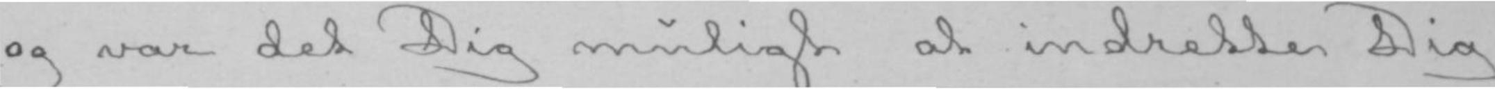og var det Dig muligt at indrette Dig
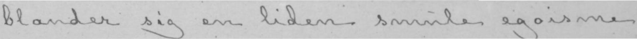blander sig en liden smule egoisme
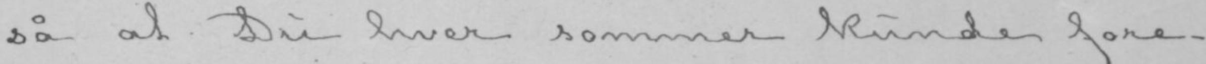så at Du hver sommer kunde fore-
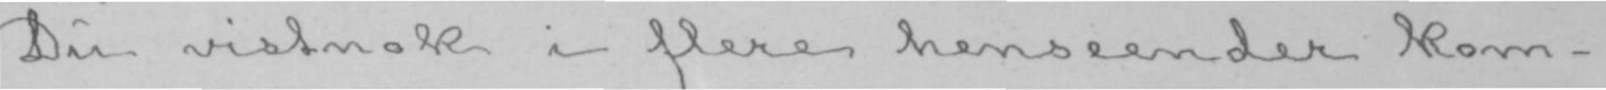Du vistnok i flere henseender kom-
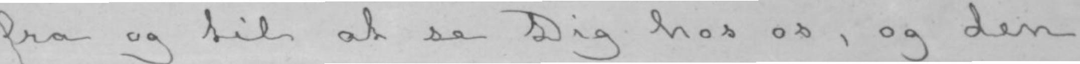fra og til at se Dig hos os, og den
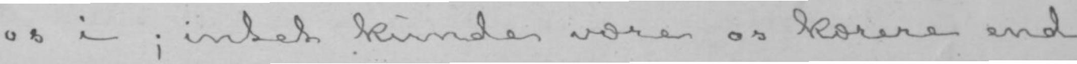os i; intet kunde være os kærere end
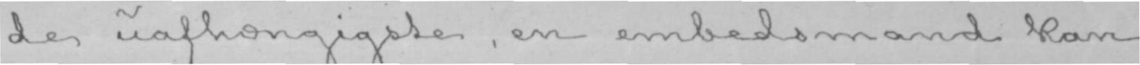de uafhængigste, en embedsmand kan
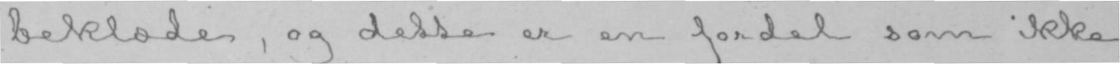beklæde, og dette er en fordel som ikke
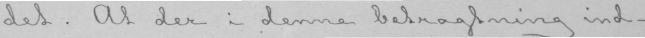det. At der i denne betragtning ind-
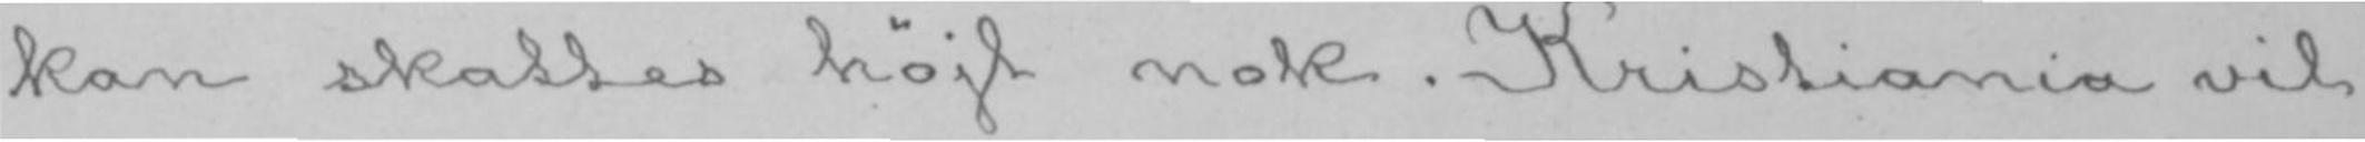kan skattes højt nok. Kristiania vil
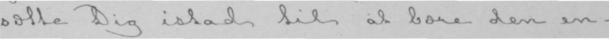sætte Dig istad til at bære den en-
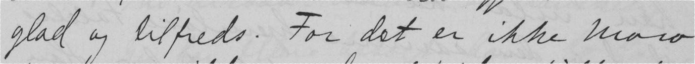glad og tilfreds. For det er ikke moro
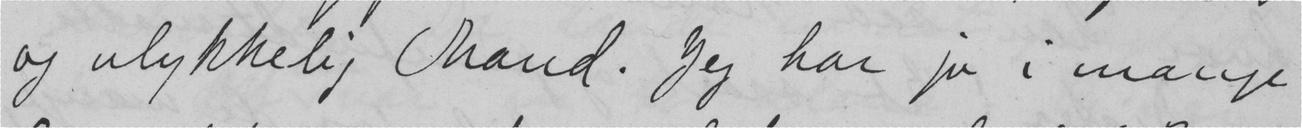og ulykkelig Mand. Jeg har jo i mange
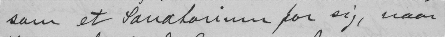som et Sanatorium for sig, naar
